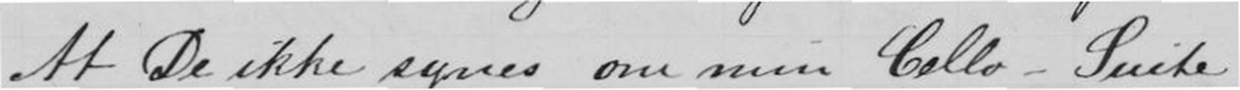At De ikke synes om min Cello-Suite
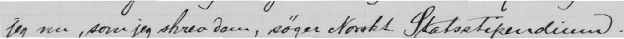jeg nu, som jeg skrev dem, søger Norskt Statsstipendium.
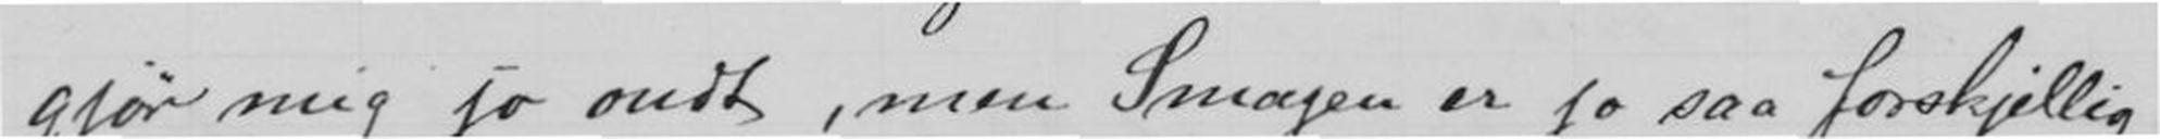gjør mig jo ondt, men Smagen er jo saa forskjellig
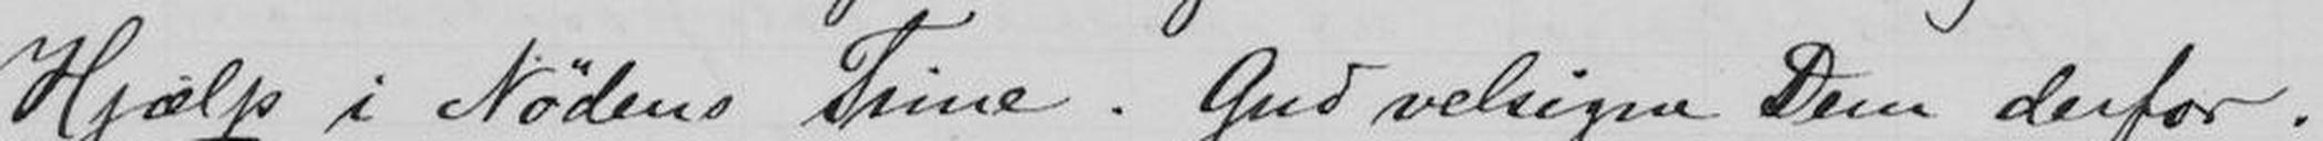Hjælp i Nødens Time. Gud velsigne Dem derfor.
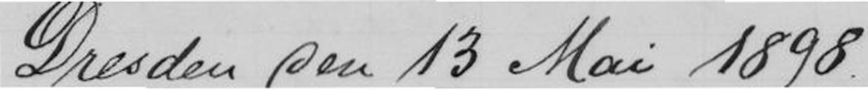Dresden den 13 Mai 1898
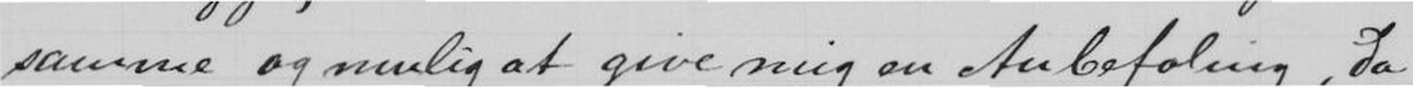samme og venlig at give mig en Anbefaling, da
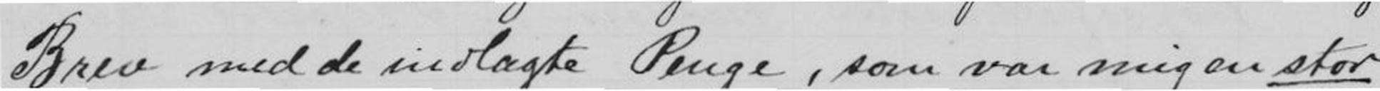Brev med de indlagte Penge, som var mig en stor
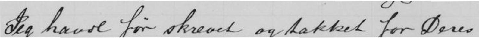Jeg havde før skrevet og takket for Deres
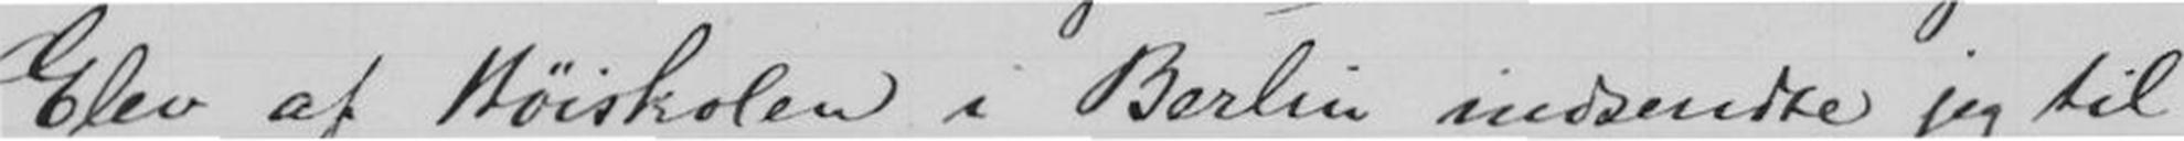Elev af Høiskolen i Berlin indsendte jeg til
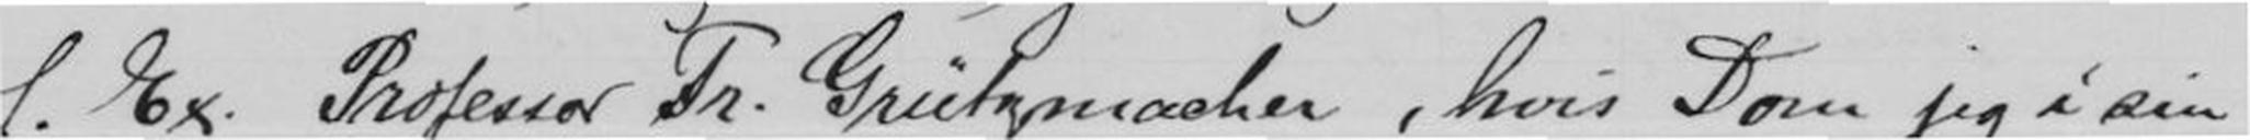f. Ex Professor Fr. Grützmacher. hvis Dom jeg i sin
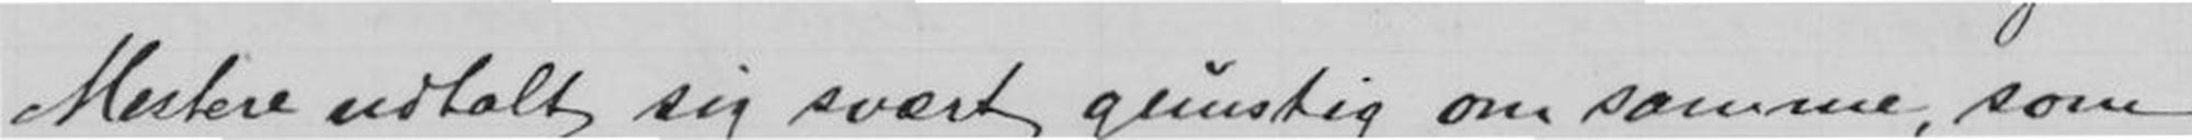Mestere udtalt sig svært gunstig om samme, som
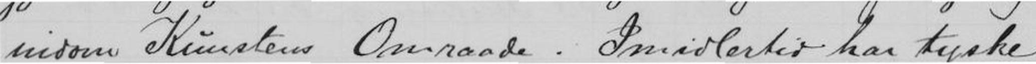indom Kunstens Omraade. Imidlertid har tyske
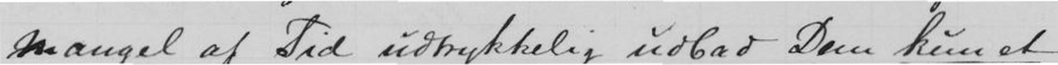mangel af Tid udtrykkelig udbad Dem kun et
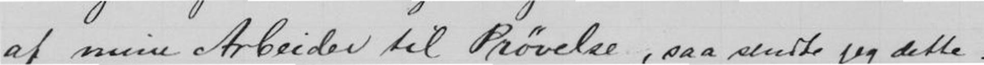af mine Arbeider til Prøvelse, saa sendte jeg dette.
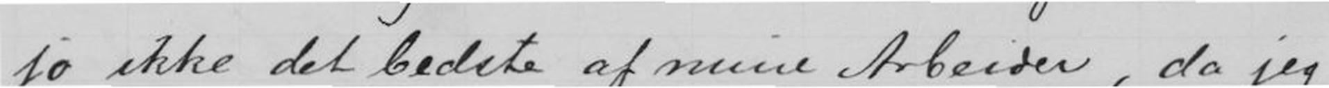jo ikke de bedste af mine Arbeider, da jeg
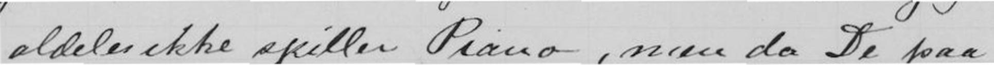aldeles ikke spiller Piano, men da De paa
